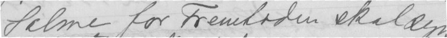Salme for Fremtiden skal syn-
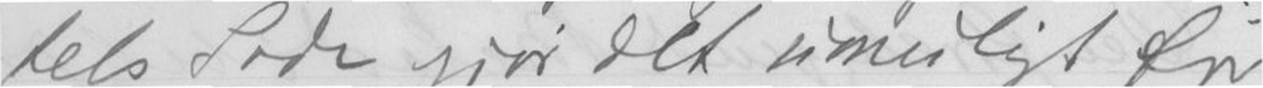tets Side gjør det umuligt for
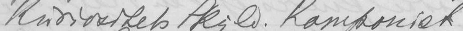Kuriositets Skyld. Komponist
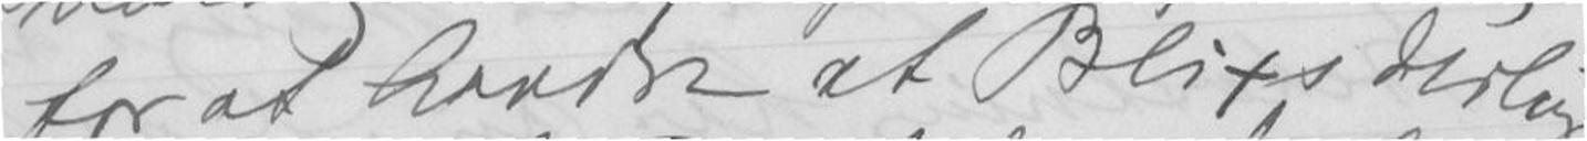for at hindre at Blixs deilige
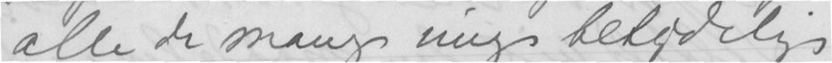alle de mange unge betydelige
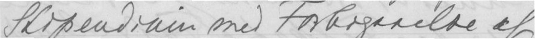Stipendium med Forbigaaelse af
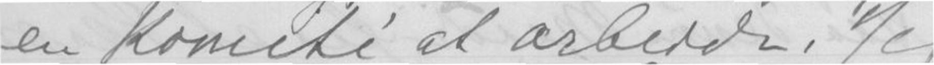en Komité at arbeide. Jeg
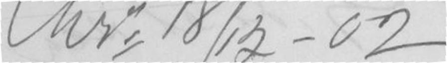Chr. 18/12-02
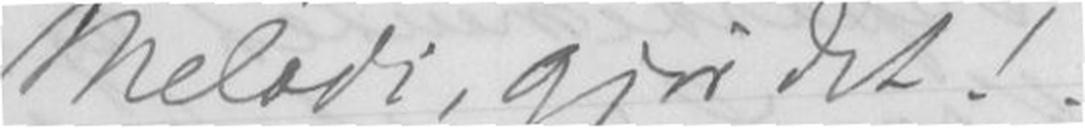Melodi, gjør det!!
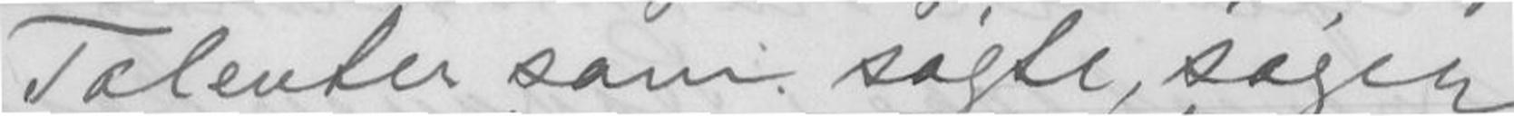Talenter som søgte, søger
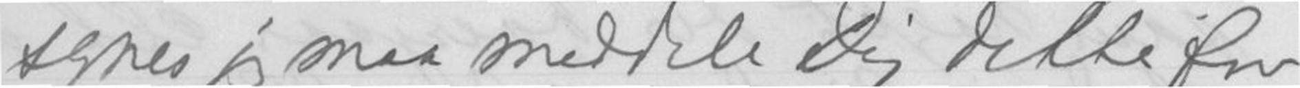synes jeg maa meddele Dig dette for
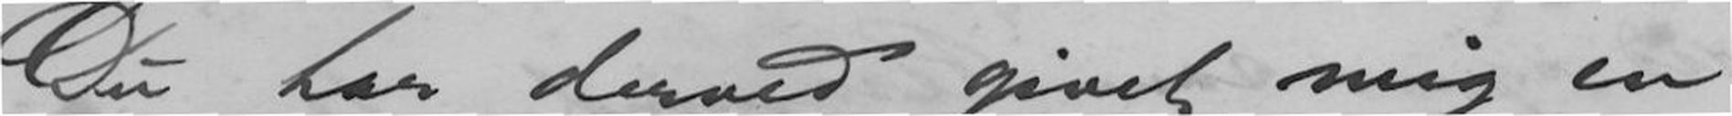Du har derved givet mig en
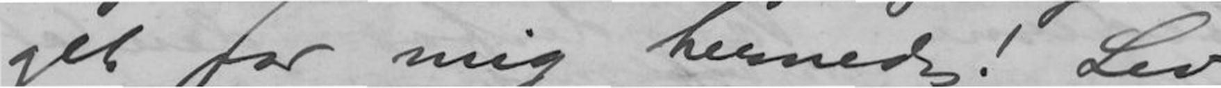get for mig hernede! Lev
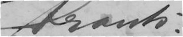Frants.
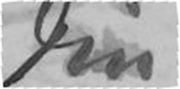Din
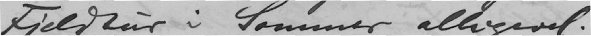Fjeldtur i Sommer alligevel.
